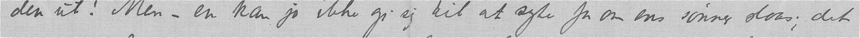den ut! Men – en kan jo ikke gi sig til at syte for om ens sønner slaas; det
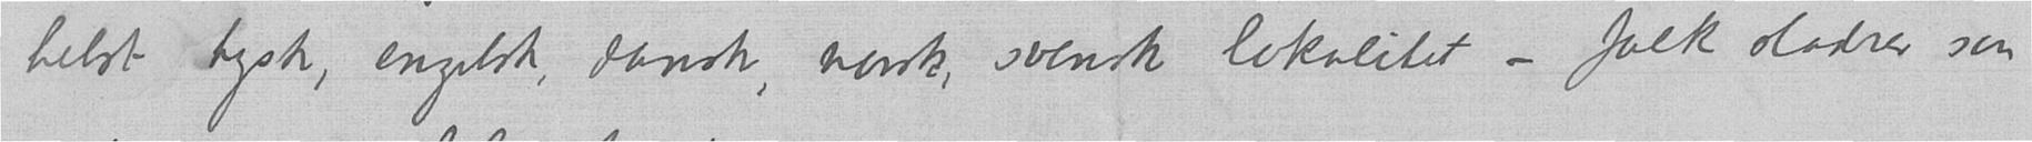helst tysk, engelsk, dansk, norsk, svensk lokalitet – folk sladrer saa
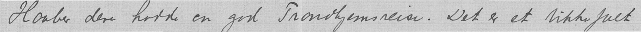Haaber dere hadde en god Trondhjemsreise. Det er et bikkefælt
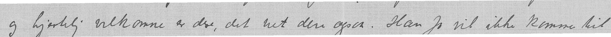og hjertelig velkomne er dere, det vet dere ogsaa. Han Jo vil ikke komme til
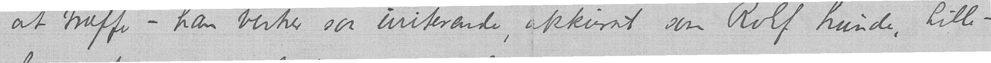at træffe – han virker saa irriterende, akkurat som Rolf Lunde, Lille-
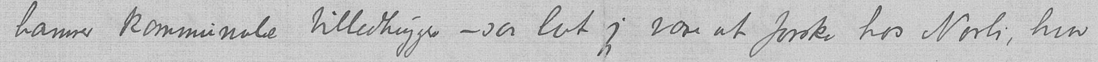hammer kommunale billedhugger – saa lot jeg være at forske hos Norli, hvor
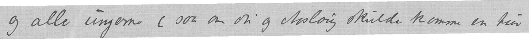og alle ungerne (saa om du og Aaslaug skulde komme en tur
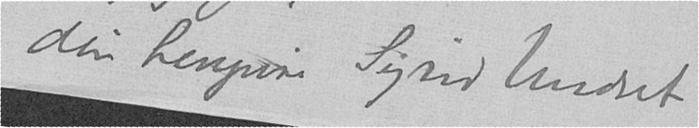din hengivne Sigrid Undset
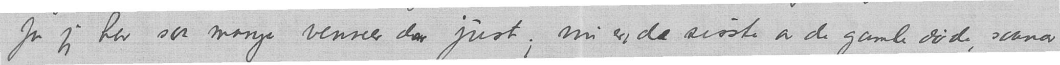for jeg har saa mange venner der just; nu er de sisste av de gamle døde, saanær
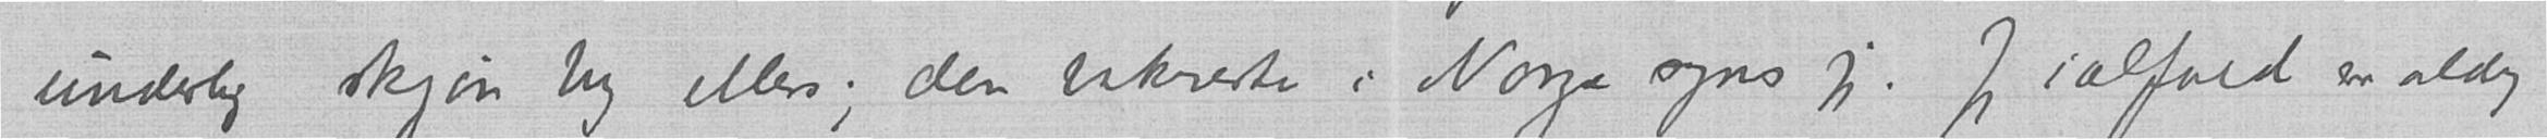underlig skjøn by ellers; den vakreste i Norge syns jeg. Jeg ialfald er aldrig
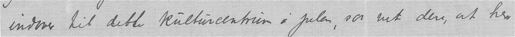indover til dette kulturcentrum i julen, saa vet dere, at her
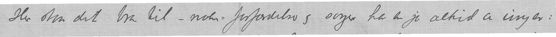Her staar det bra til – noen forfærdelser og sorger har en jo altid av unger:
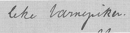leke barnepiker.
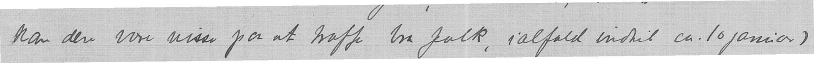kan dere være visse paa at træffe bra folk, ialfald indtil ca. 10 januar)
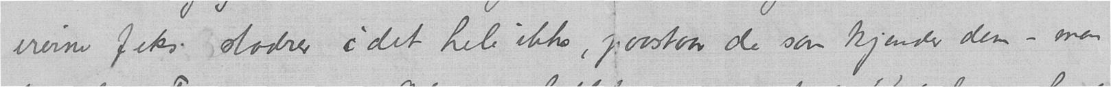irerne feks. sladrer i det hele ikke, paastaar de som kjender dem – men
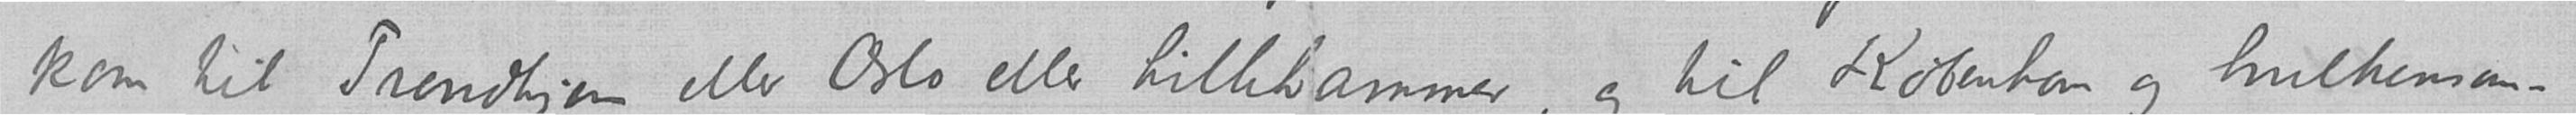kom til Trondhjem eller Oslo eller Lillehammer, og til København og hvilkensom-
